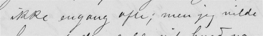ikke engang ofte; men jeg vilde
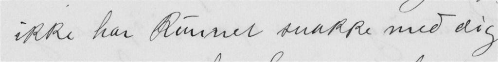ikke har Kunnet snakke med dig
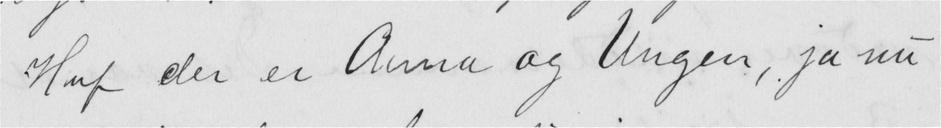Huf der er Anna og Ungen, ja nu
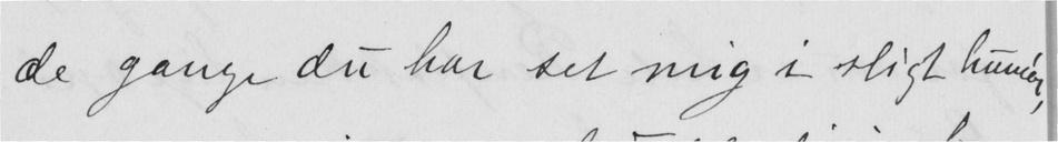de gange du har set mig i sligt humør,
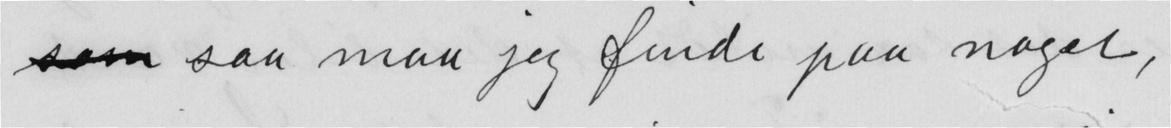som saa maa jeg finde paa noget,
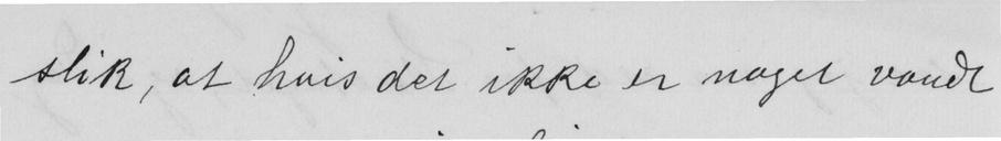slik, at hvis det ikke er noget vondt
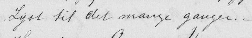Lyst til det mange ganger.-
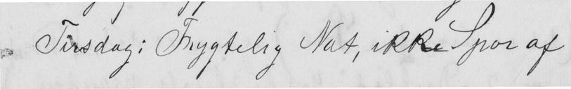Tirsdags: Frygtelig Nat, ikke Spor af
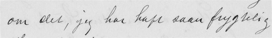om det, jeg har haft saan frygtelig
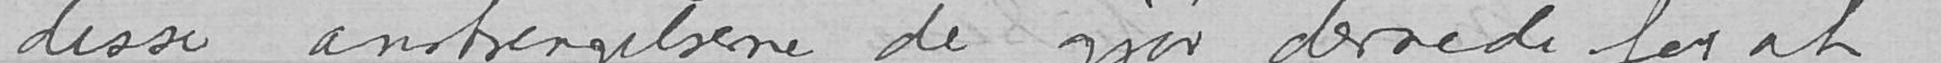disse anstrengelserne de gjør der nede for at
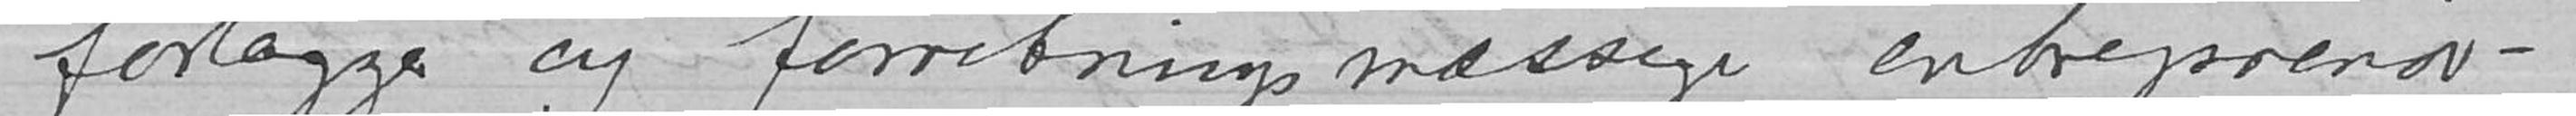forlægger og forretningsmæssige entreprenør –.
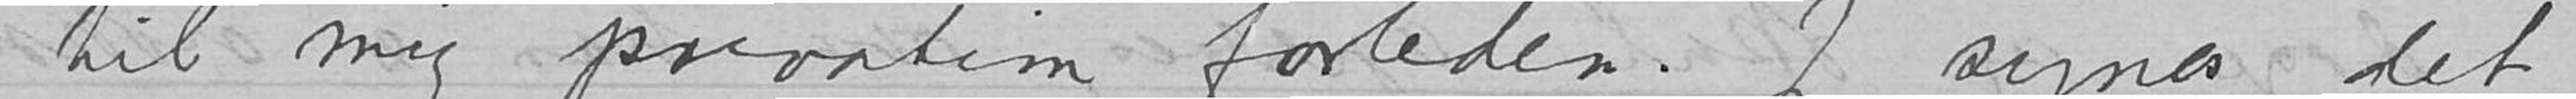til mig privatim forleden. Jeg synes, det
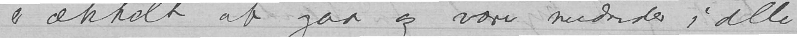er ækkelt at gaa og være medvider i alle
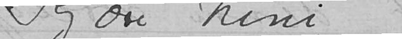Kjære Nini,
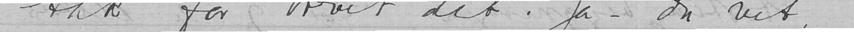Tak for brevet dit. Ja – du vet,
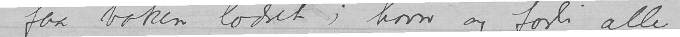faa boken lodset i havn og forbi alle
